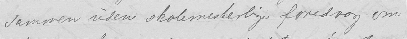sammen uden skolemesterlige foredrag om
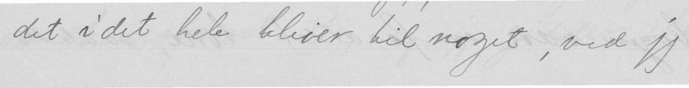det i det hele bliver til noget, ved jeg
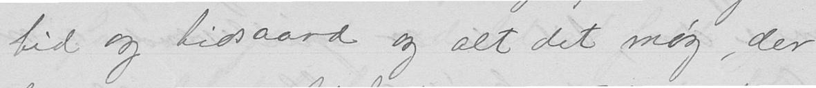tid og tidsaand og alt det møg, der
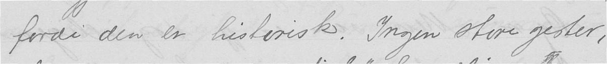fordi den er historisk. Ingen store gester,
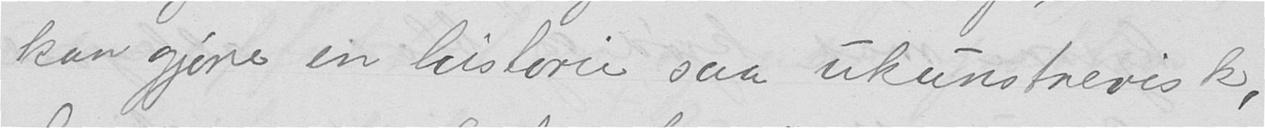kan gjøre en historie saa ukunstnerisk,
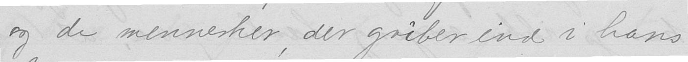og de mennesker, der griber ind i hans
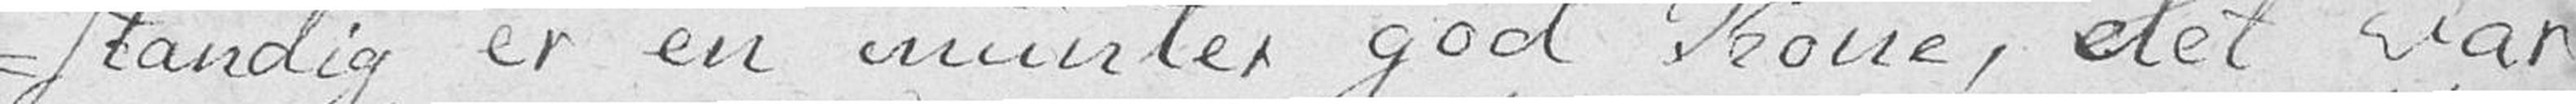-standig er en munter god Kone, det var
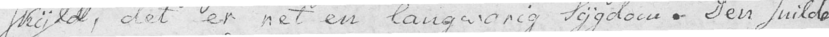skyld, det er net en langvarig Sygdom. Den snilde
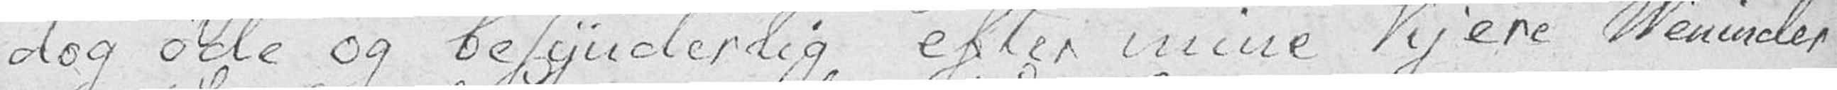dog øde og besynderlig efter mine kjere Veninder
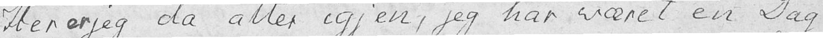Her er jeg da atter igjen, jeg har været en Dag
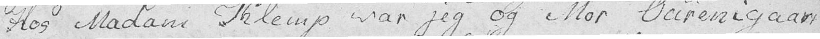Hos Madam Klemp var jeg og Mor Ouren igaar,
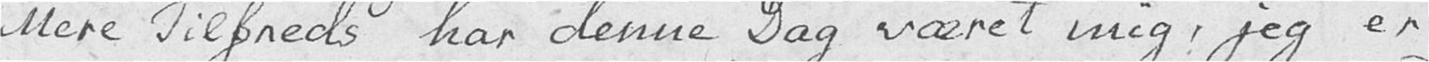Mere Tilfreds har denne Dag været mig, jeg er
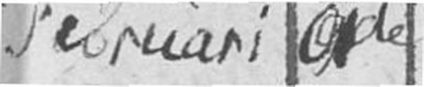Februari 01de
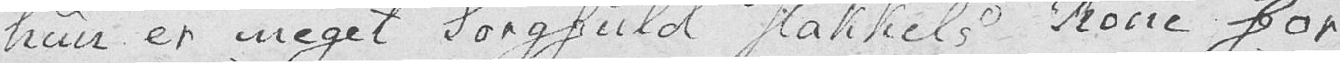hun er meget Sorgfuld stakkels Kone for
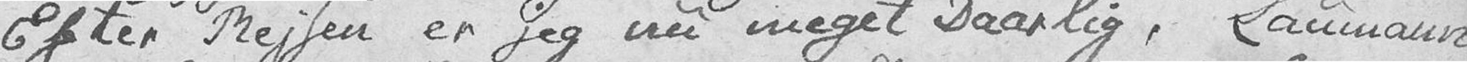Efter Rejsen er jeg nu meget Daarlig, Laumann
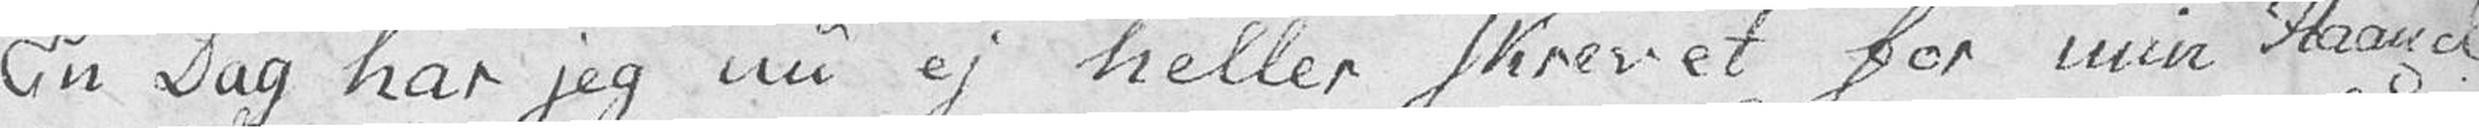En Dag har jeg nu ej heller skrevet for min Haand
